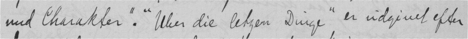und Charakter". "Uber die letzen Dinge" er udgivet efter
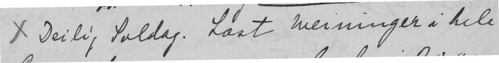x Deilig Soldag. Læst Weininger i hele
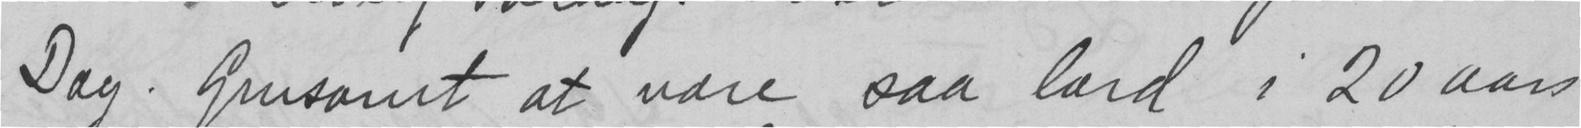Dag. Grusomt at være saa lærd i 20 Aars
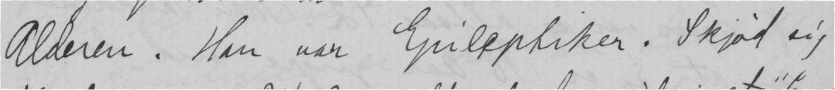Alderen. Han var Epileptiker. Skjød sig
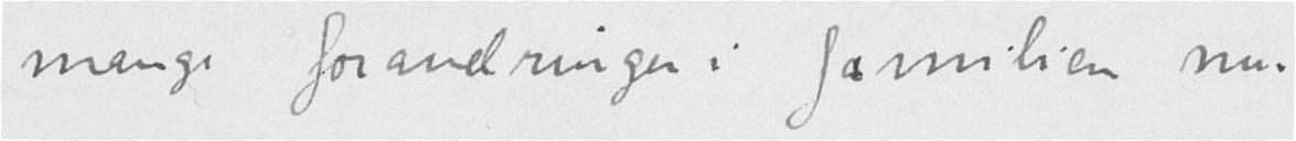mange forandringer i familien nu.
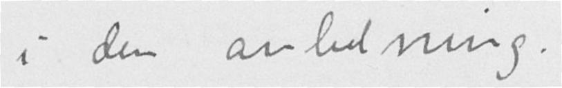i den anledning.
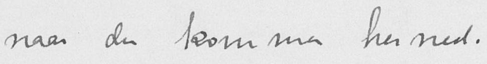naar du kommer herned.
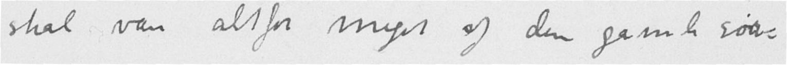skal være altfor meget af den gamle sør-
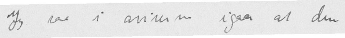Jeg saa i aviserne i gaar at din
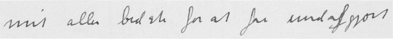mit aller bedste for at faa undafgjort
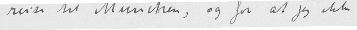reise til München, og for at jeg ikke
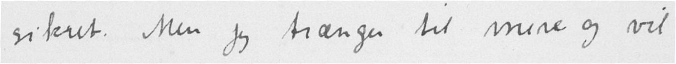sikret. Men jeg trænger til mere og vil
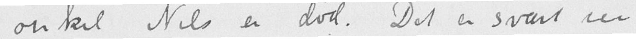onkel Nils er død. Det er svært saa
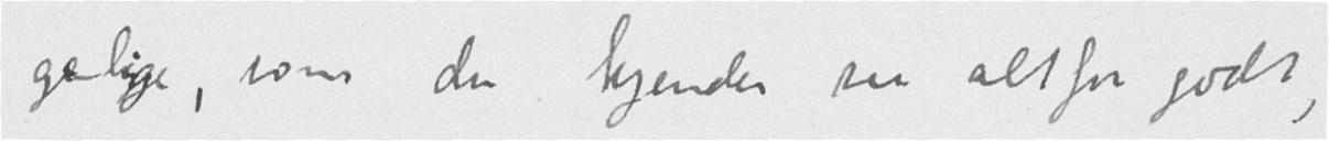gelige, som du kjender saa altfor godt,
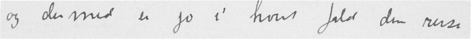og dermed er jo i hvert fald din reise
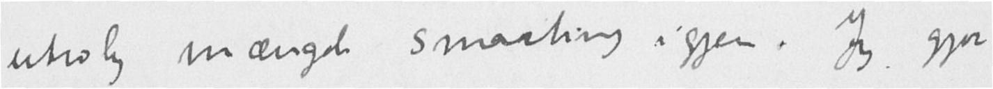utrolig mængde smaating igjen. Jeg gjør
